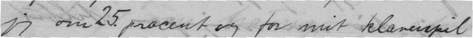jeg om 25 procent, og for mit klaverspil
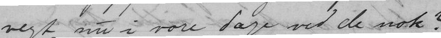vegt nu i vore dage ved de nok?
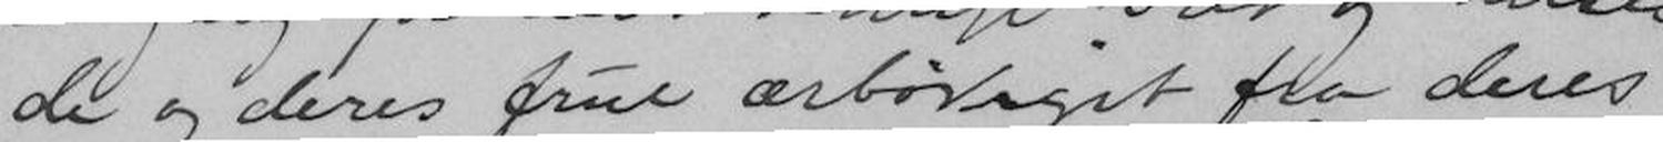de og deres frue ærbødigst fra deres
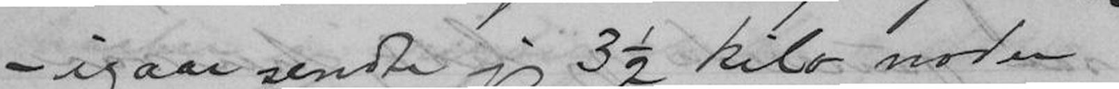- igaar sendte jeg 3½ kilo noder
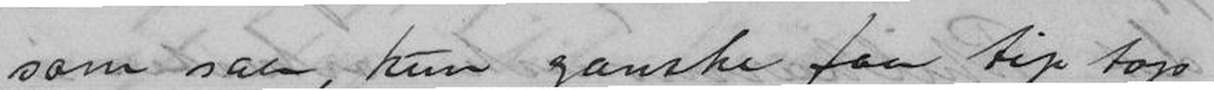som saa, kun ganske faa tip top
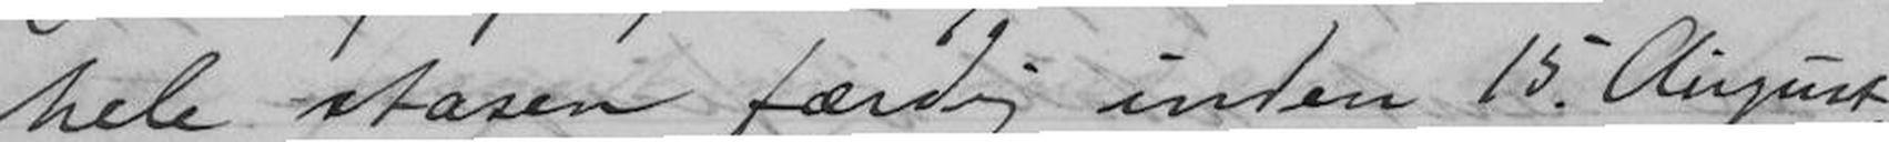hele stasen færdig inden 15 August.
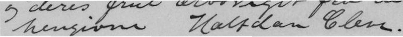hengivne Halvdan Cleve.
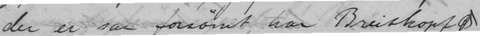der er saa forsømt, har Breitkopfs &
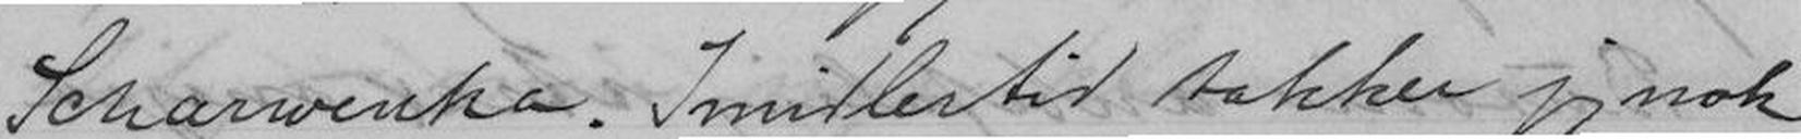Scharwenka. Imidlertid takker jeg nok
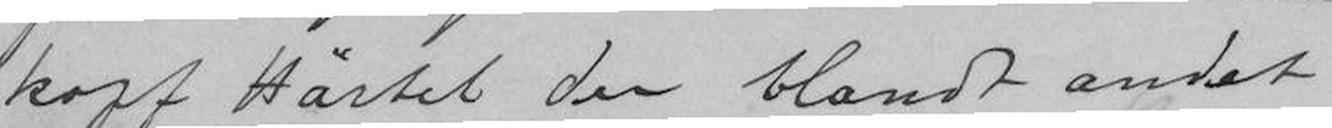kopf Härtel den blandt andet
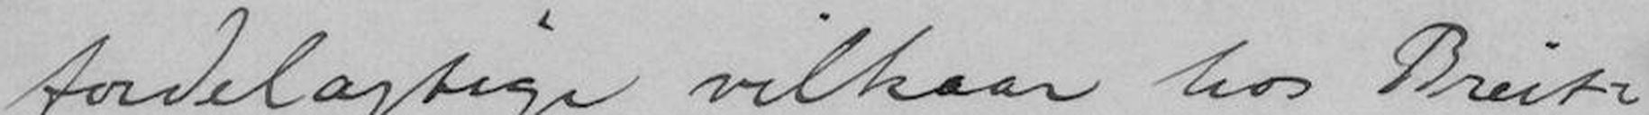fordelagtig vilkaar hos Breit-
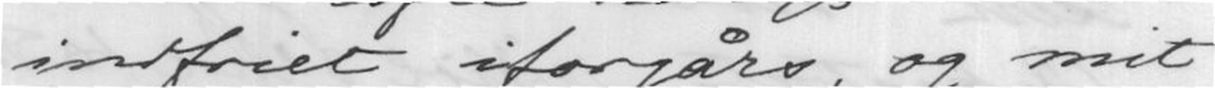indfriet iforgårs, og mit
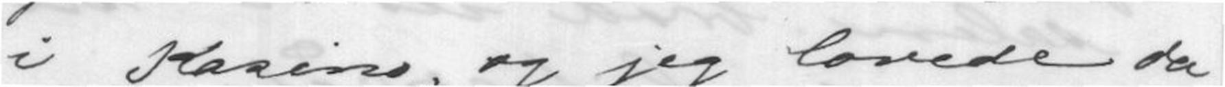i Kasino; og jeg lovede da
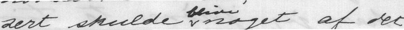sert skulde noget af det
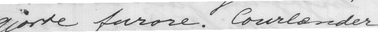gjorde furore Courlænder
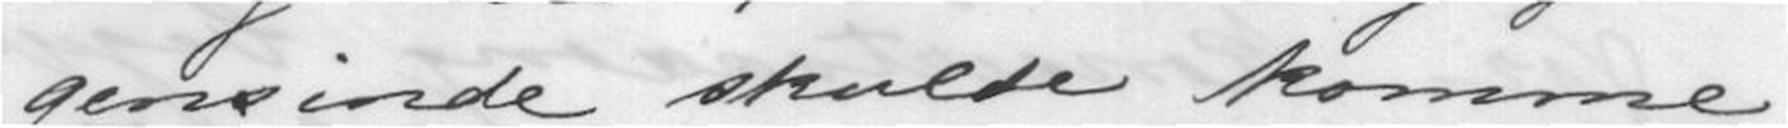gensinde skulde komme
